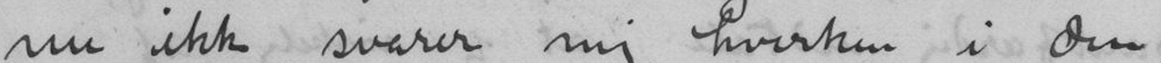nu ikke svarer mig hverken i den
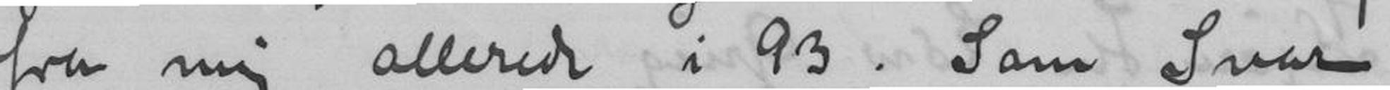fra mig allerede i 93. Som Svar
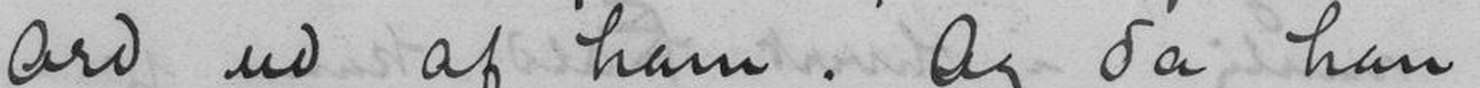Ord ud af ham. Og da han
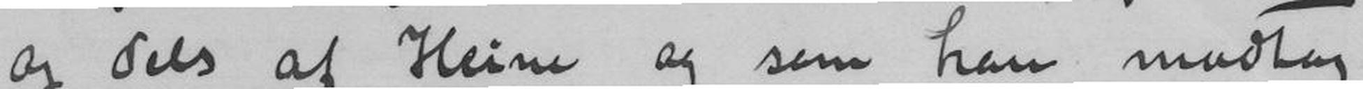og dels af Heine og som han modtog
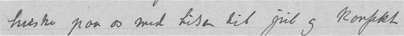huske paa os med hilsen til jul og konfekt
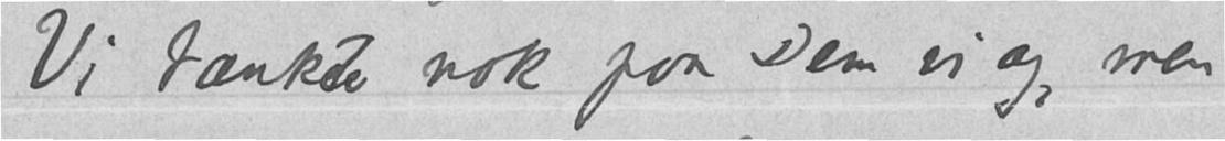Vi tænkte nok paa Dem vi og, men
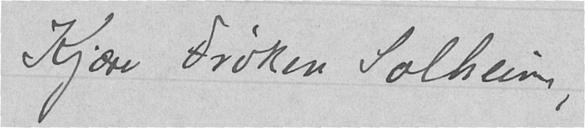Kjære Frøken Solheim,
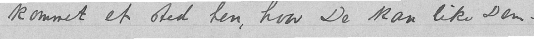kommet et sted hen, hvor De kan like Dem –
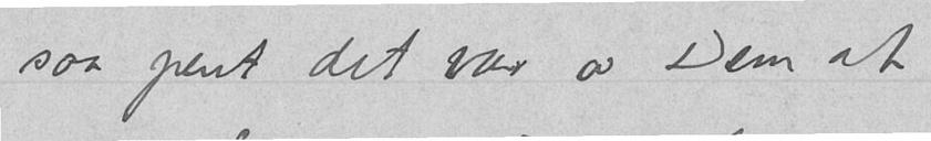saa pent det var av Dem at
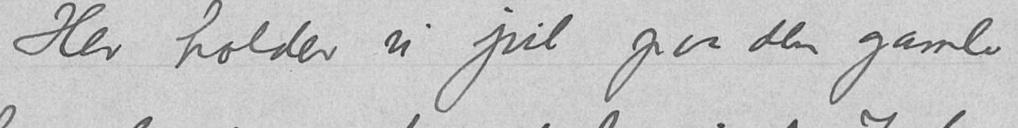Her holder vi jul paa den gamle
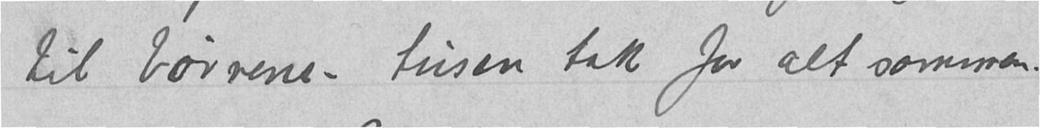til børnene – tusen tak for alt sammen.
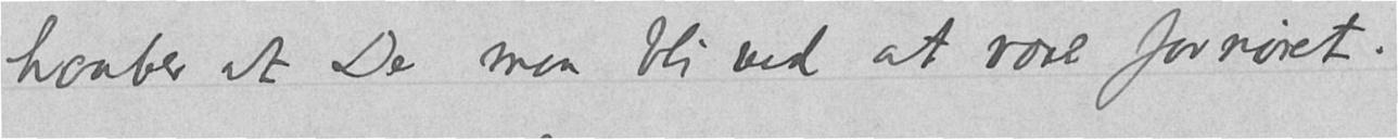haaber at De maa bli ved at være fornøiet.
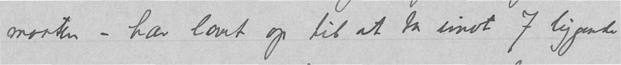maaten – har lavet op til at ta imot 7 liggende
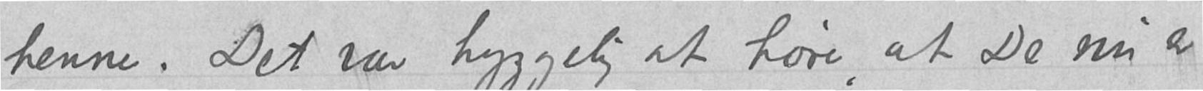henne. Det var hyggelig at høre, at De nu er
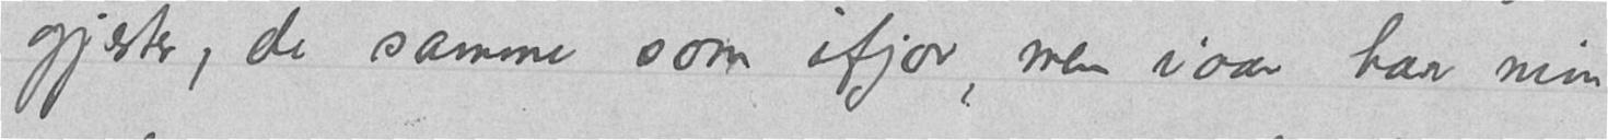gjester, de samme som ifjor, men iaar har min
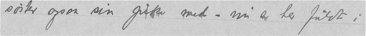søster ogsaa sin pike med – nu er her fuldt i
